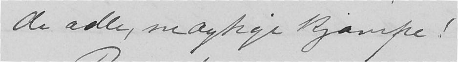de ædle, megtige kjæmpe!
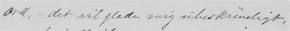ord, - det vil glæde mig ubeskriveligt,
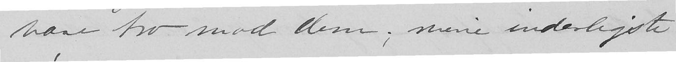være tro mod dem; mine inderligste
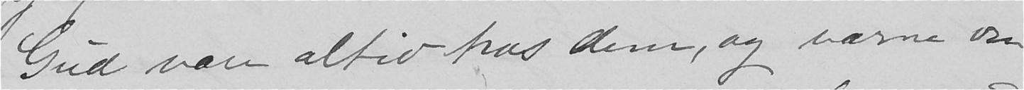Gud være altid hos dem, og værne om
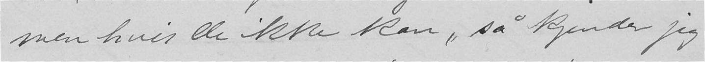men hvis de ikke kan, så kjender jeg
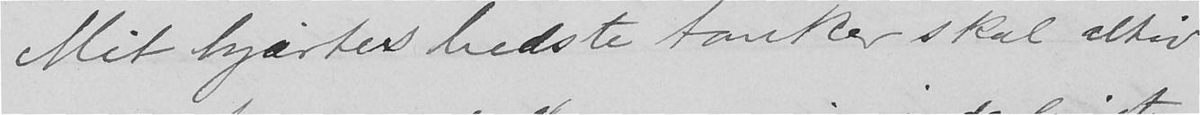Mit hjærtes bedste tanker skal altid
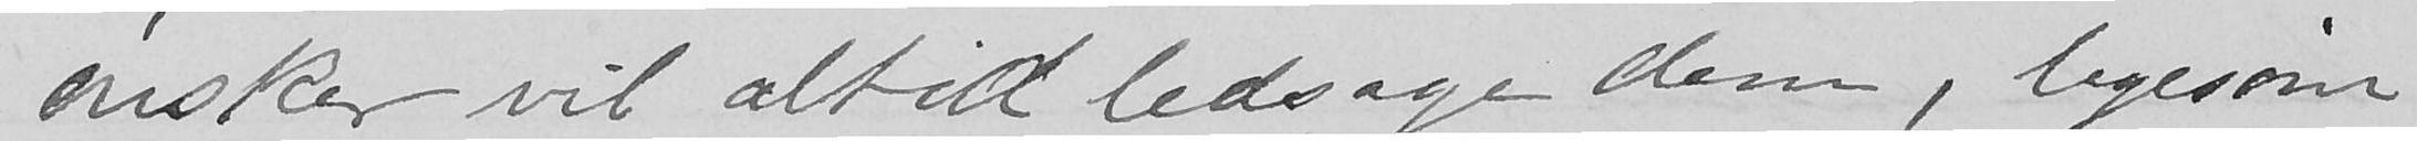ønsker vil altid ledsage dem, ligesom
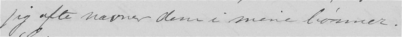Jeg ofte nævner dem i mine bønner.
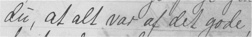du, at alt var af det gode,
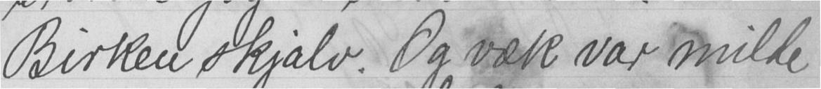Birken skjalv. Og væk var milde
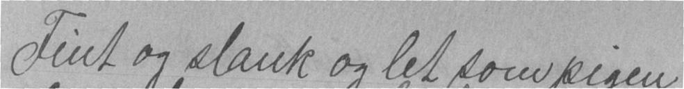Fint og slank og let som pigen,
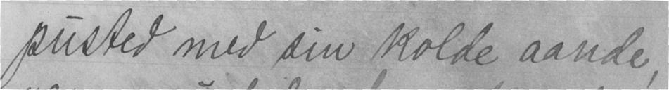pusted med sin kolde aande,
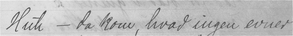Huh - da kom, hvad ingen evner
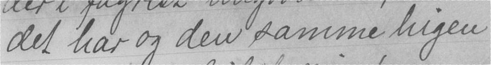det har og den samme higen
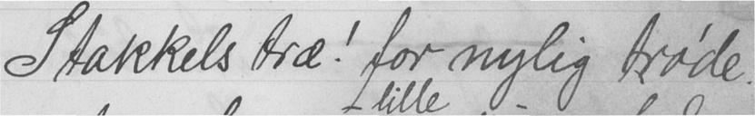Stakkels træ! for nylig trøde.
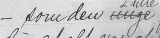- som den unge
